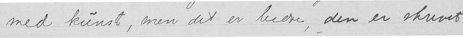med kunst, men det er bedre, den er skrevet
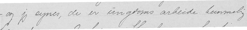- og jeg synes, de er ungdoms arbeide temmelig
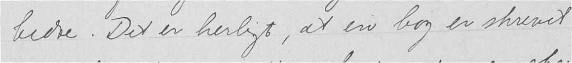bedre. Det er herligt, at en bog er skrevet
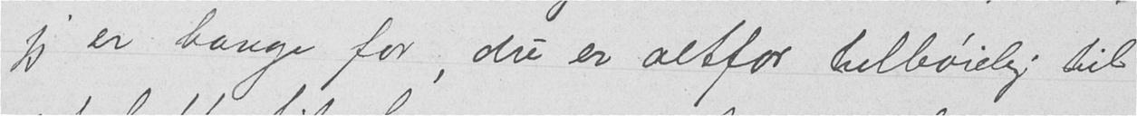jeg er bange for, du er altfor tilbøielig til
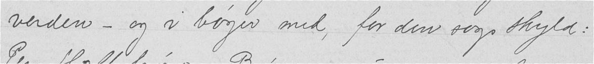verden - og i bøger med, for den sags skyld:
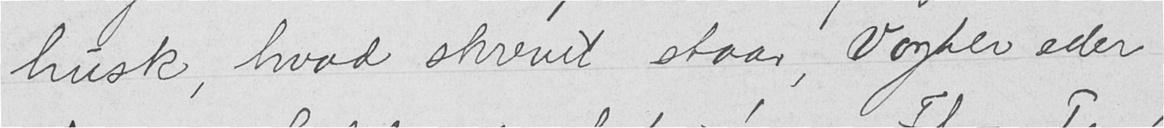husk, hvad skrevet staar, Vogter eder
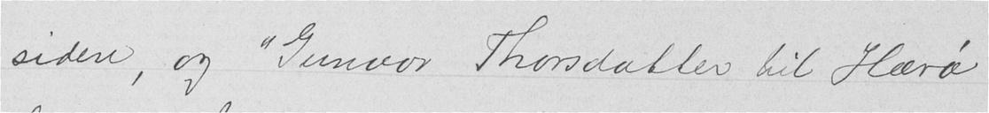siden, og "Gunvor Thorsdatter til Hærø"
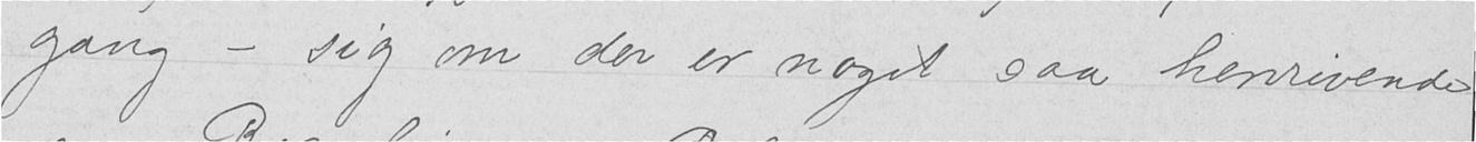gang - sig om der er noget saa henrivende
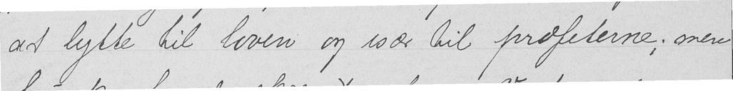at lytte til loven og især til profeterne; men
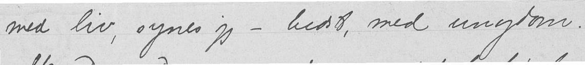med liv, synes jeg - bedst, med ungdom.
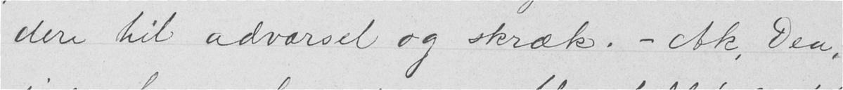dere til advarsel og skræk. - Ak, Dea,
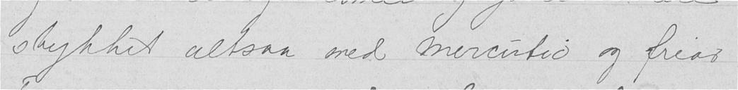stykket altsaa med Mercutio og friar
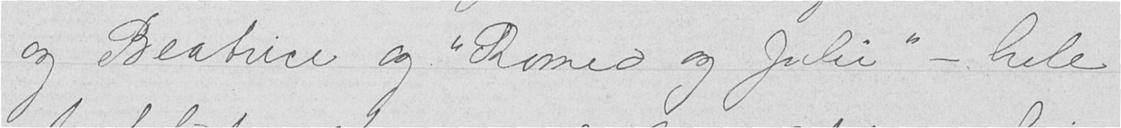og Beatrice og "Romeo og Julie" - hele
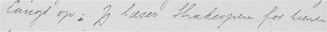langt op. Jeg læser Shakespeare for tiende
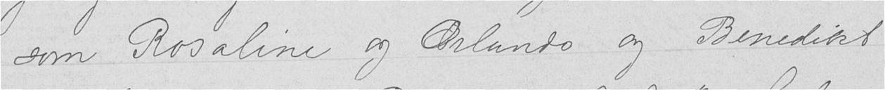som Rosaline og Orlando og Benedikt
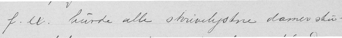f.ex. burde alle skrivelystne damer stu-
